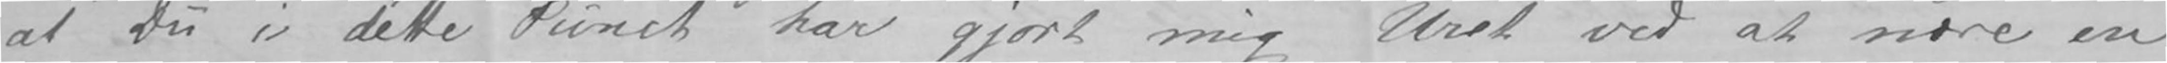at Du i dette Punct har gjort mig Uret ved at nære en
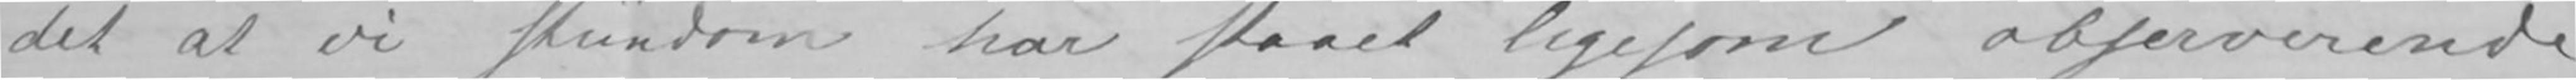det at vi stundom har staaet ligesom observerende
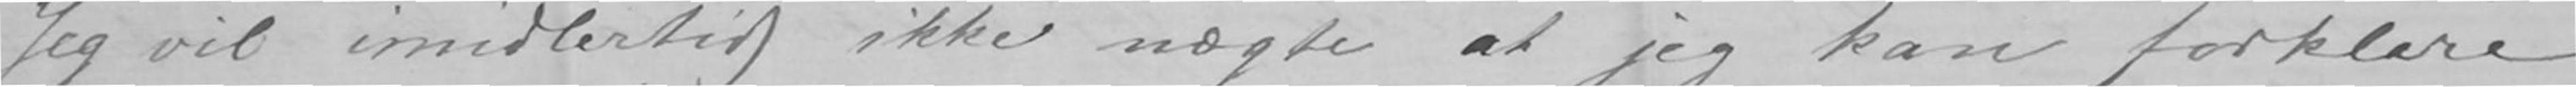Jeg vil imidlertid ikke nægte at jeg kan forklare
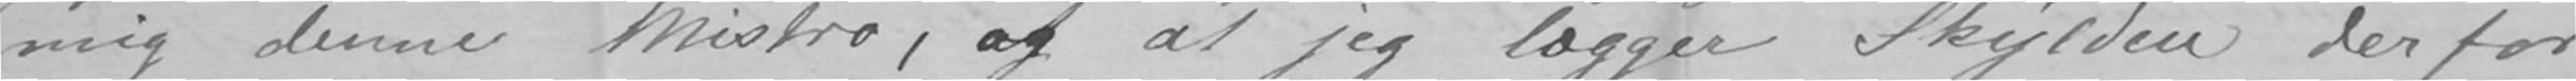mig denne Mistro, ogat at jeg lægger Skylden derfor
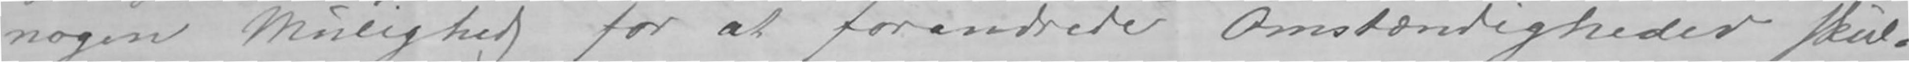nogen Mulighed for at forandrede Omstændigheder skul-
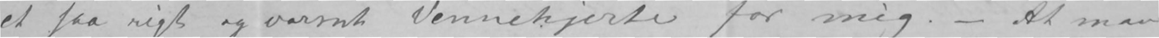et saa rigt og varmt Vennehjerte for mig.- At man
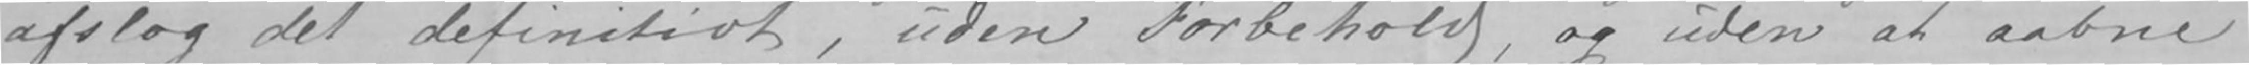afslog det definitivt, uden Forbehold, og uden at aabne
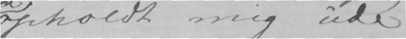opholdt mig ude
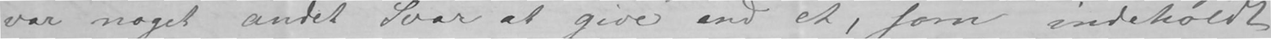var noget andet Svar at give end et, som indeholdt
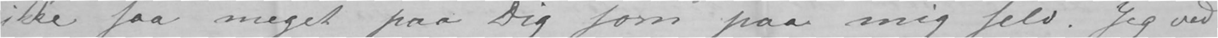ikke saa meget paa Dig som paa mig selv. Jeg ved
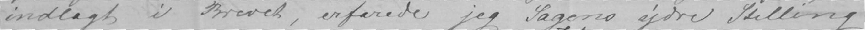indlagt i Brevet, erfarede jeg Sagens ydre Stilling
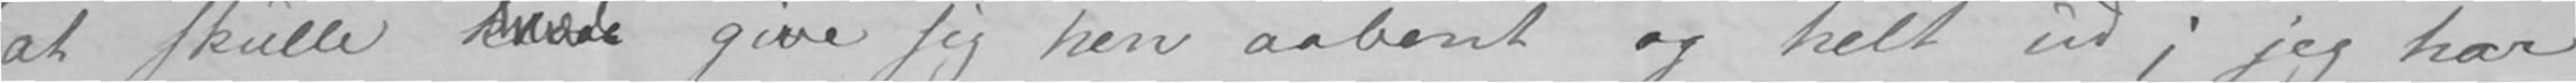at skulle knæle give sig hen aabent og helt ud; jeg har
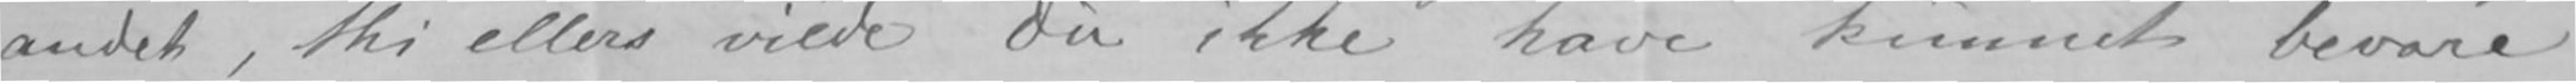andet, thi ellers vilde Du ikke have kunnet bevare
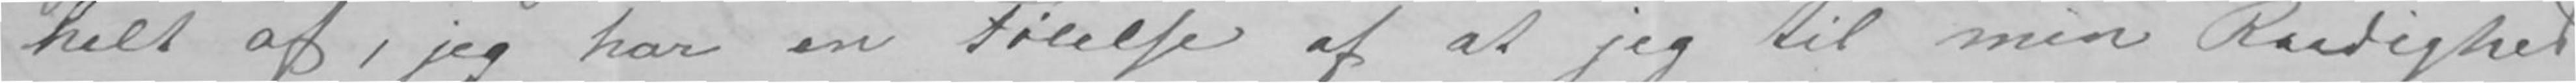helt af, jeg har en Følelse af at jeg til min Raadighed
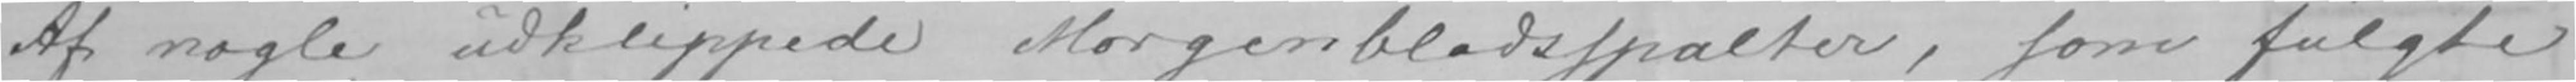Af nogle udklippede Morgenbladsspalter, som fulgte
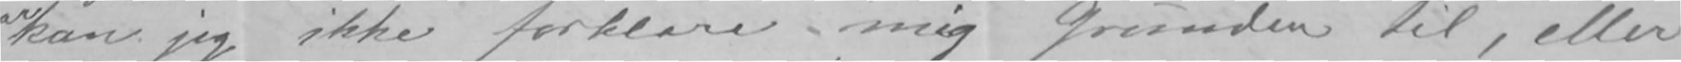kan jeg ikke forklare mig Grunden til, eller
